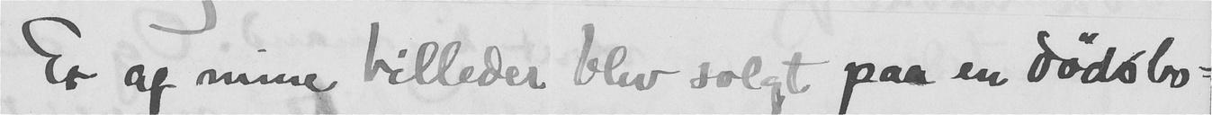Et af mine billeder blev solgt paa en dødsbo-
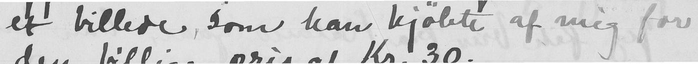et billede, som han kjøbte af mig for
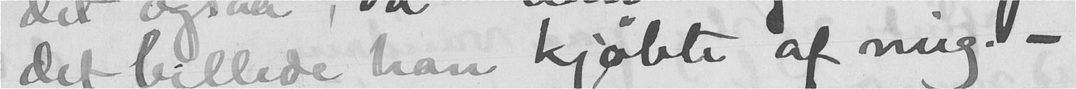det billede han kjøbte af mig. -
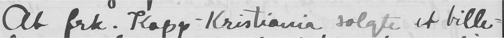At frk. Kapp-Kristiania solgte et bille-
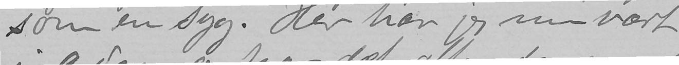som en syg. Her har jeg nu vært
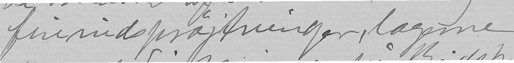finindspøjtninger, lægerne
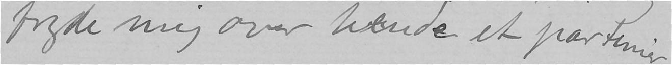fryde mig over hende et par timer
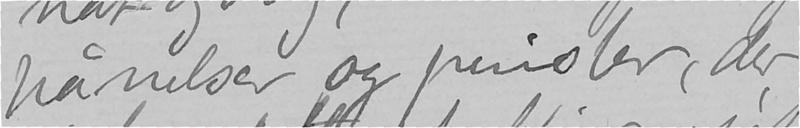hånelser og pinsler, der
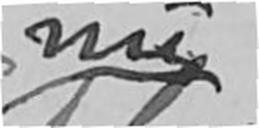nu
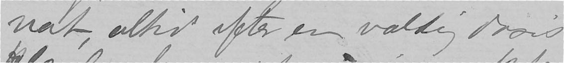nat, altid efter en vældig dosis
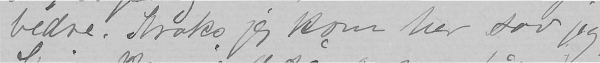bedre. Straks jeg kom her sov jeg.
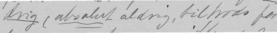drig, absolut aldrig, tiltrods for
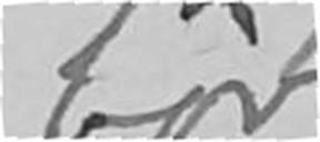bor
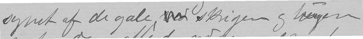synet af de gale, ved skrigen og hujen
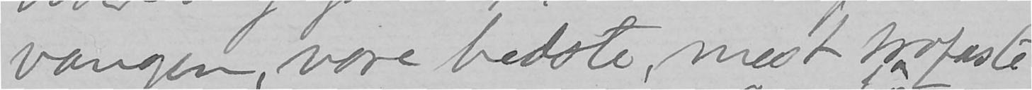vangen, vore bedste, mest trofaste
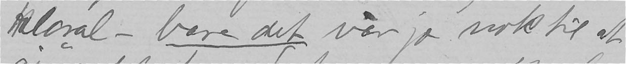kloral - bare det var jo nok til at
